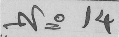No 14
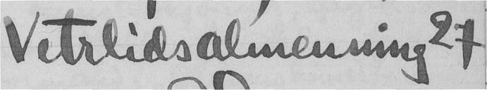Vetrlidsalmenning 27
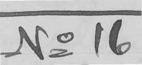No 16
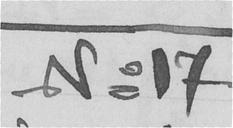No 17
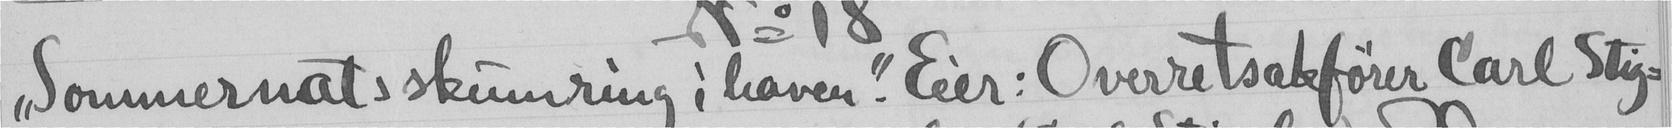"Sommernatsskumring i haven". Eier: Overretsakfører Carl Stig-
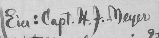Eier: Capt. H.J. Meyer
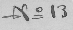No 13
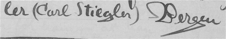ler (Carl Stiegler) Bergen
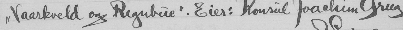"Vaarkveld og Regnbue". Eier: Konsul Joachim Grieg
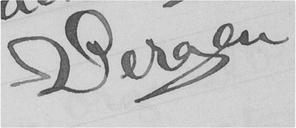Bergen
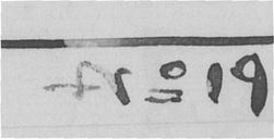No 19
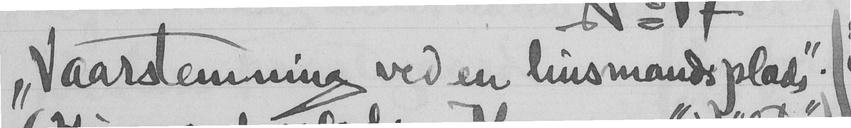"Vaarstemning ved en husmandsplads".
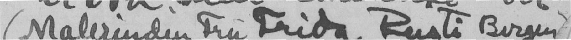(Malerinden Fru Frida Rusti Bergen)
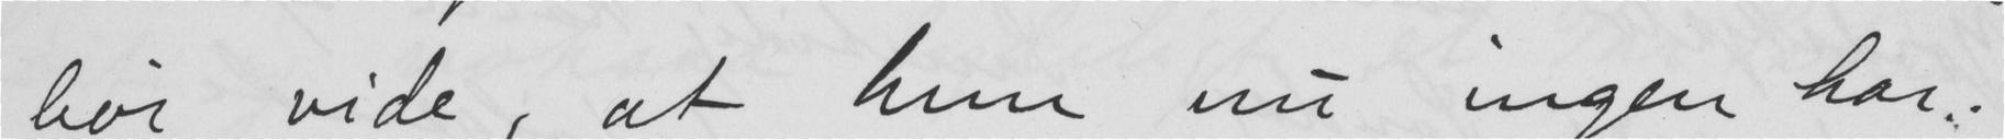bør vide, at hun nu ingen har,
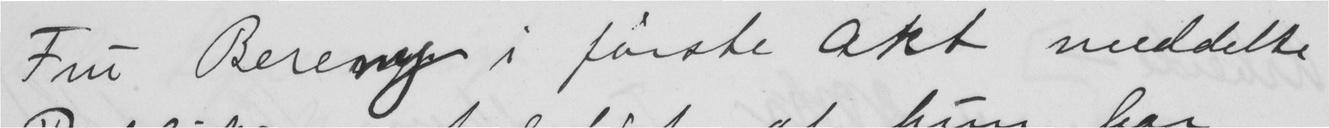Fru Bereny i første Akt meddelte
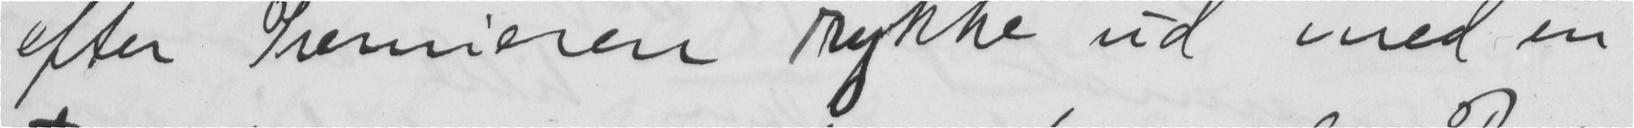efter Premieren rykke ud med en
